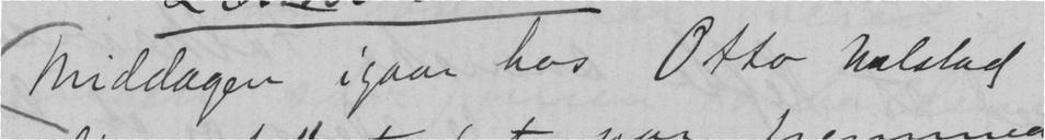Middagen igaar hos Otto Valstad
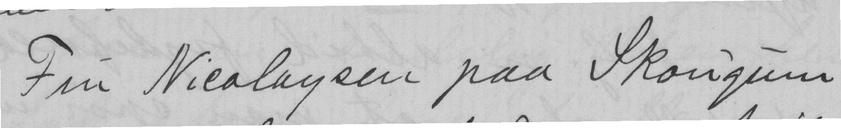Fru Nicolaysen paa Skougum
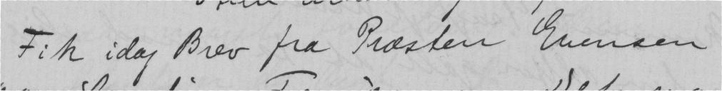Fik idag Brev fra Præsten Evensen
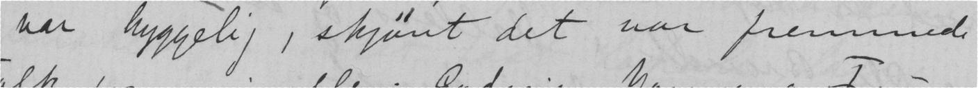var hyggelig, skjønt det var fremmede
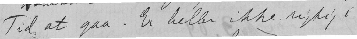Tid at gaa. Er heller ikke rigtig i
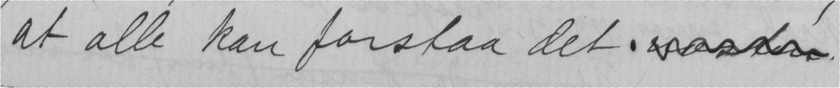at alle kan forstaa det.
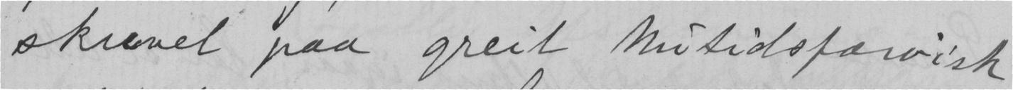skrevet paa greit nutidsfærøisk
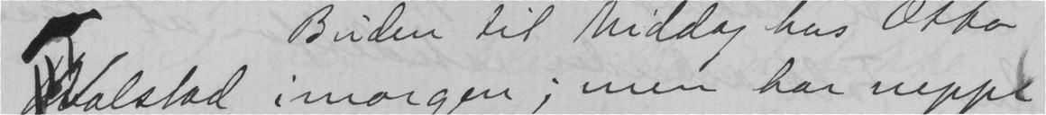Valstad imorgen; men har neppe
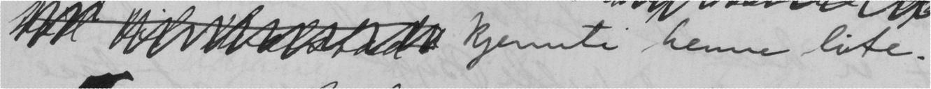kjennte henne lite.
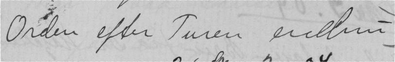Orden efter Turen endnu
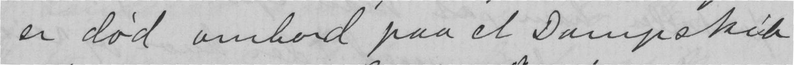er død ombord paa et Dampskib
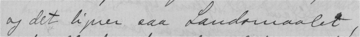og det ligner saa Landsmaalet,
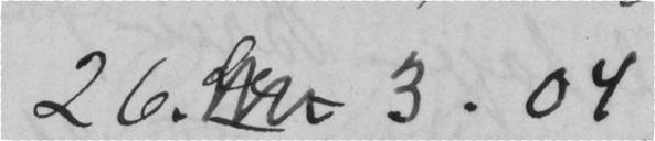26. 3.04
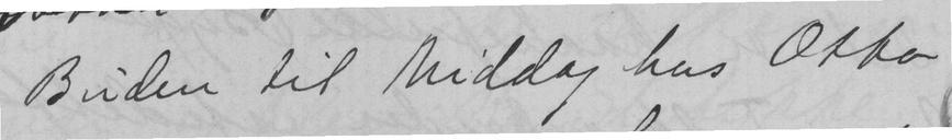Buden til Middag hos Otto
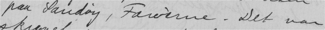paa Sandøy, Færøerne. Det var
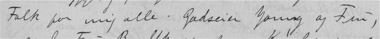Folk for mig alle. Godseier Young og Fru,
